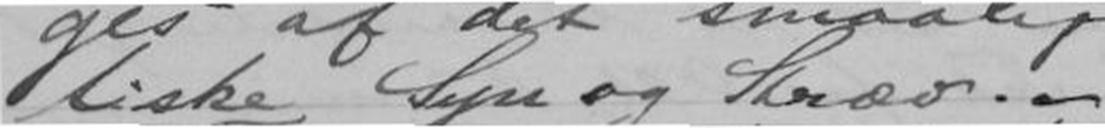tiske Syn og Stræv. -
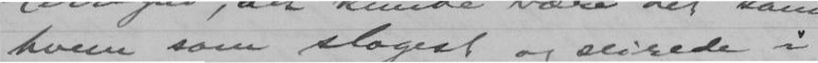hvem som slogest og seirede i
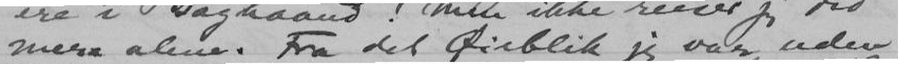mere alene. Fra det Øieblik jeg var uden
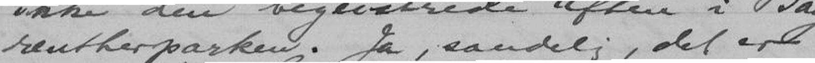reutherparken. Ja, sandelig, det er
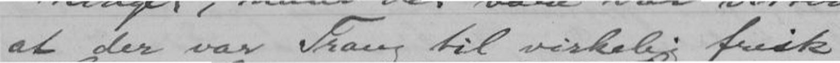at der var Trang til virkelig frisk
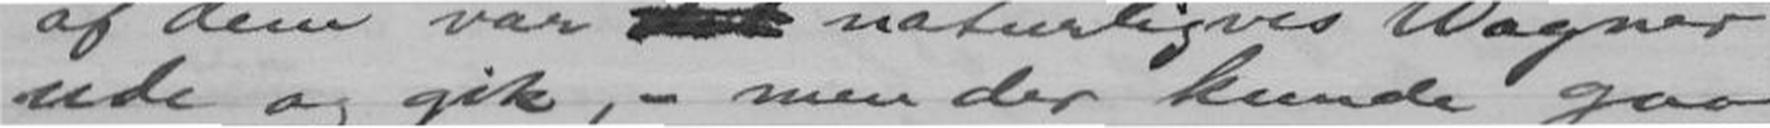ude og gik, - men der kunde gaa
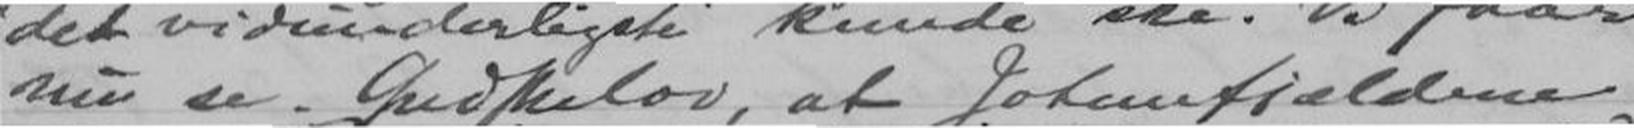nu se. Gudskelov, at Jotunfjælderne
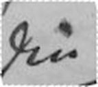Din
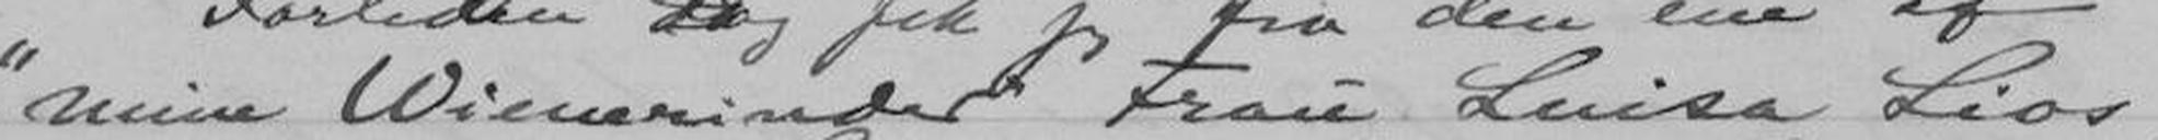"mine Wienerinder" Frau Luisa Lios,
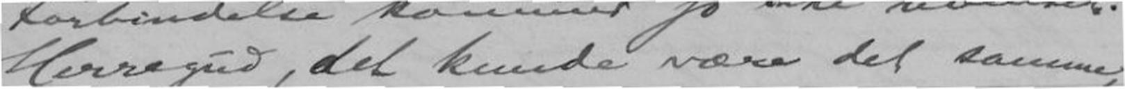Herregud, det kunde være det samme,
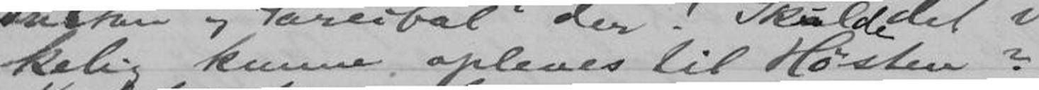kelig kunne opleves til Høsten?
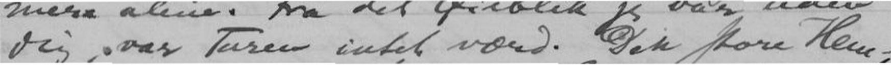Dig, var Turen intet værd. Den store Hem-
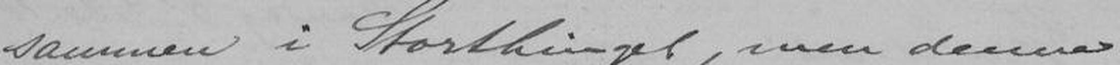sammen i Storthinget, men denne
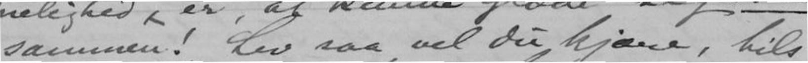sammen! Lev saa vel Du Kjære, hils
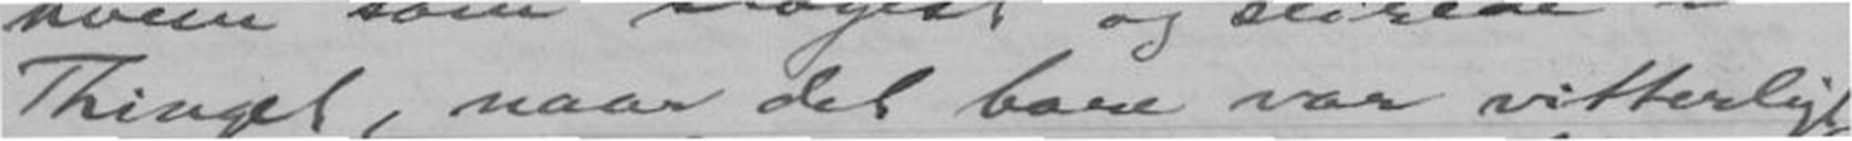Thinget, naar det bare var vitterligt
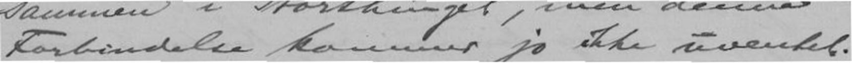Forbindelse kommer jo ikke uventet.
